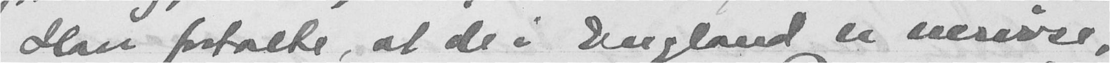Han fortalte, at de i England er nervøse,
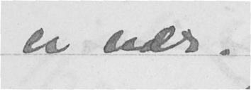er nær.
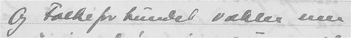Og Folkeforbundet vakler mer
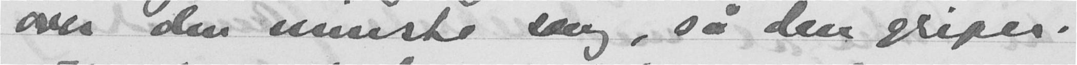over den minste sang, så den gripes.
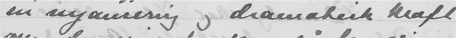en nyansering og dramatisk kraft
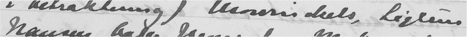i betraktning) Mowinckels, Sigrun
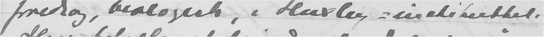foredrag, biologisk, i Huxly-instituttet.
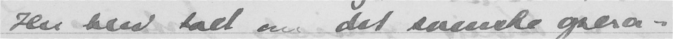Her blev talt om det svenske opera-
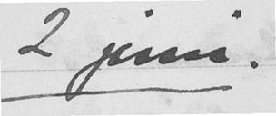2 juni.
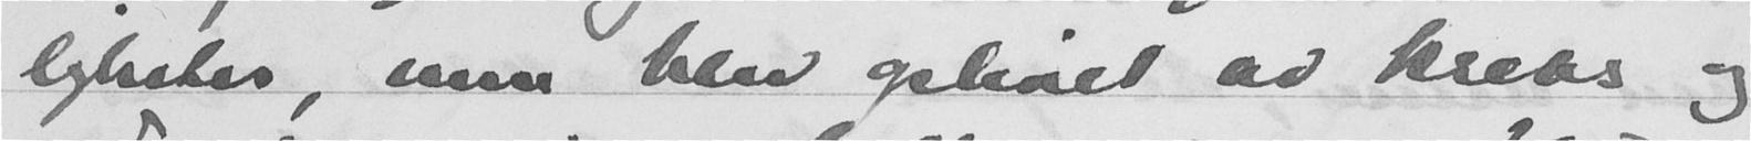ligheter, men blev oplivet av krebs og
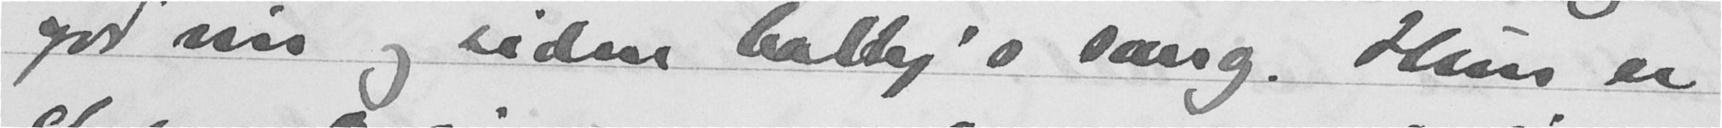god vin og siden Cally's sang. Hun er
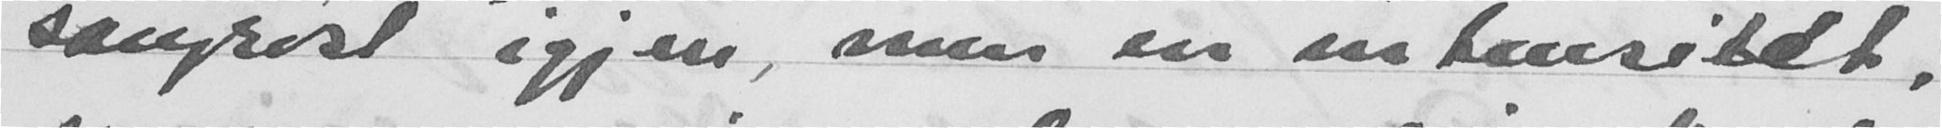sangrøst igjen, men en intensitet,
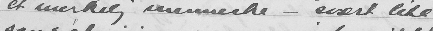et merkelig menneske - svært lite
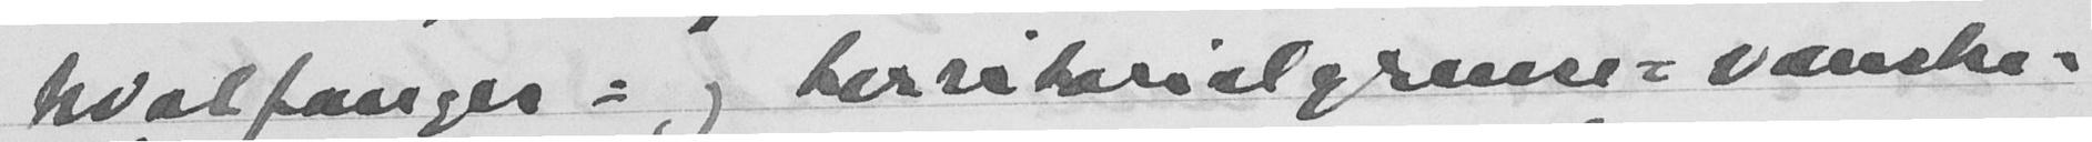hvalfangst- og territorialgrense-vanske-
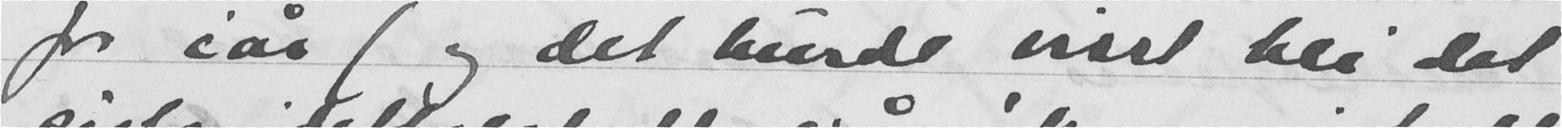for iår (og det burde vist bli det
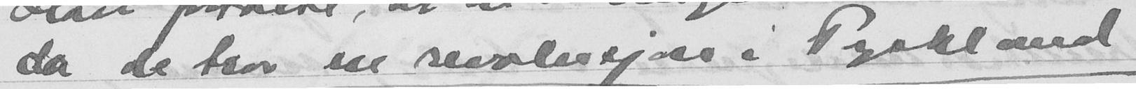da de tror en revolusjon i Tyskland
